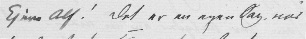Kjere Alf. Det er en egen Sag, når
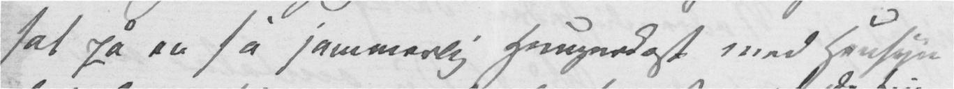sat på en så jammerlig Hungerkost med Hensyn
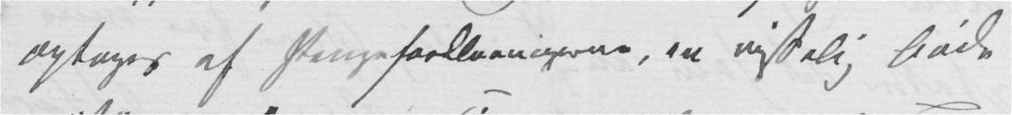optages af Pengeforklaringerne, en visselig både
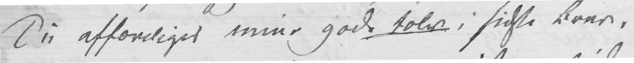Du affærdiger mine gode tolv i sidste Brev,
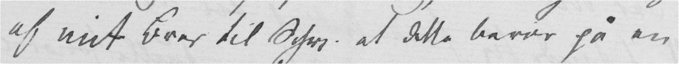af mit Brev til Schw. at dette beror på en
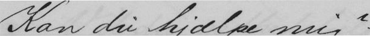Kan du hjælpe mig ?
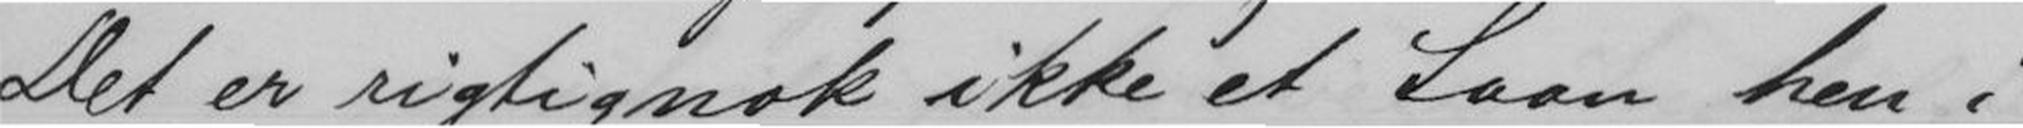Det er rigtignok ikke et Laan hen i
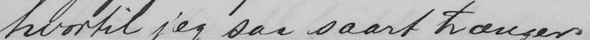hvortil jeg saa saart trænger
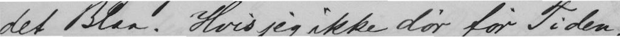det Blaa. Hvis jeg ikke dør før Tiden,
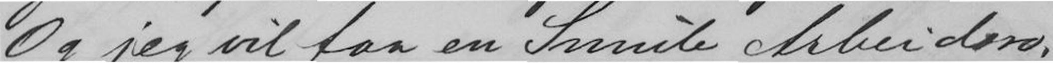Og jeg vil faa en Smule Arbeidsro,
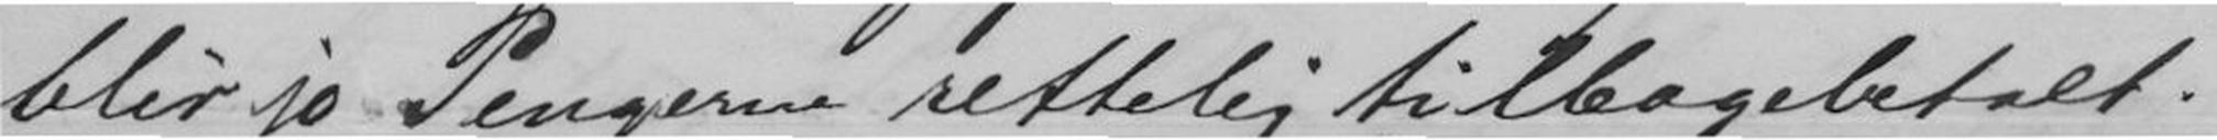blir jo Pengene rettelig tilbagebetalt.
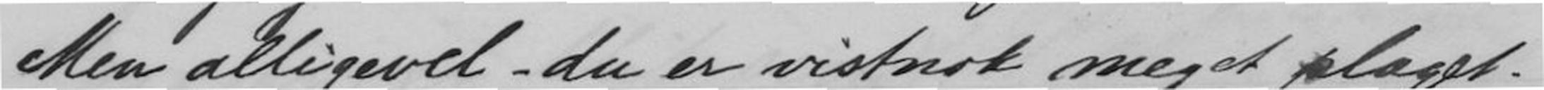Men alligevel - du er vistnok meget plaget.
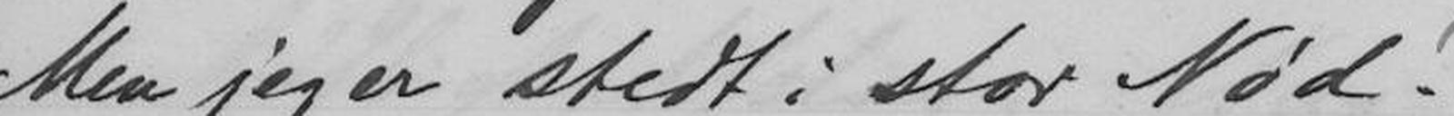Men jeg er stedt i stor Nød !
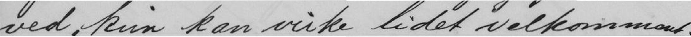ved, kun kan virke lidet velkommen.
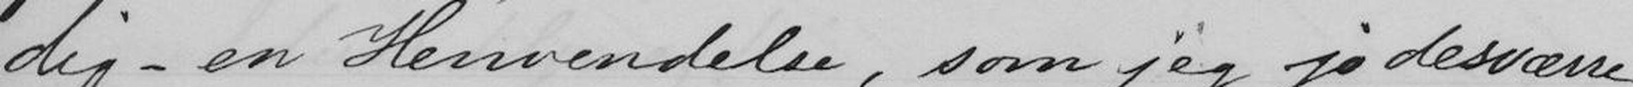dig - en Henvendelse, som jeg jo desværre
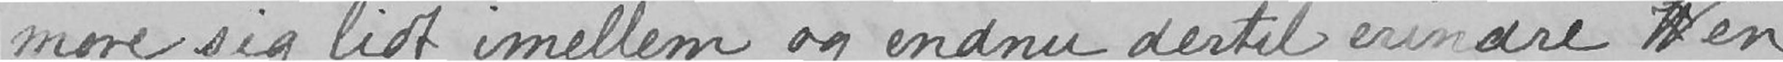more sig lidt imellem og endnu dertil erindre Wen
